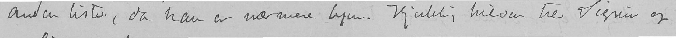anden liste, da han er nærmere byen. Hjertelig hilsen til Sigrun og
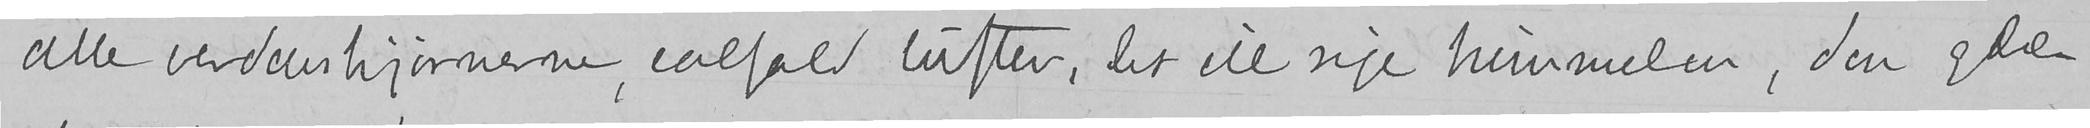alle verdenshjørnerne, ialfald luften, det vil sige himmelen, den glæ-
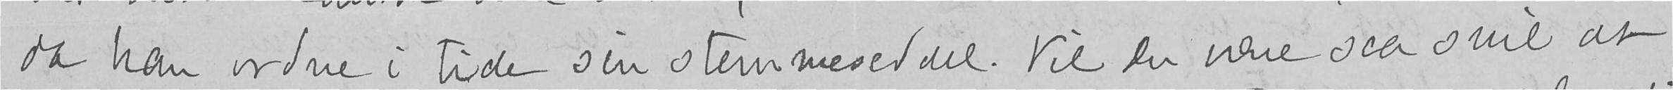da kan ordne i tide sin stemmeseddel. Vil du være saa snil at
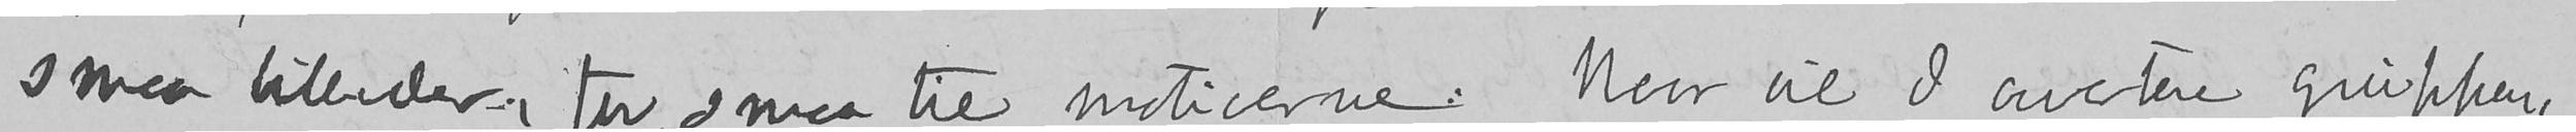smaa billeder, for smaa til motiverne. Naar vil I avertere gruppen,
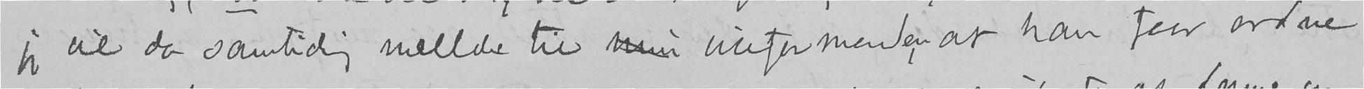jeg vil da samtidig mellde til min bileformanden at han faar ordne
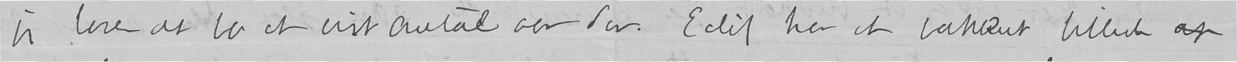jeg lover at bo et vist antal aar der. Eilif har et vakkert billede af
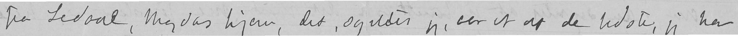fra Ledaal, Magdas hjem, det, syntes jeg, var et af de bedste, jeg har
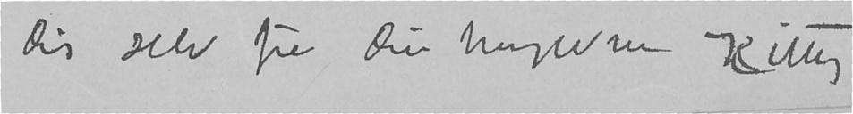dig selv fra din hengivne Kitty.
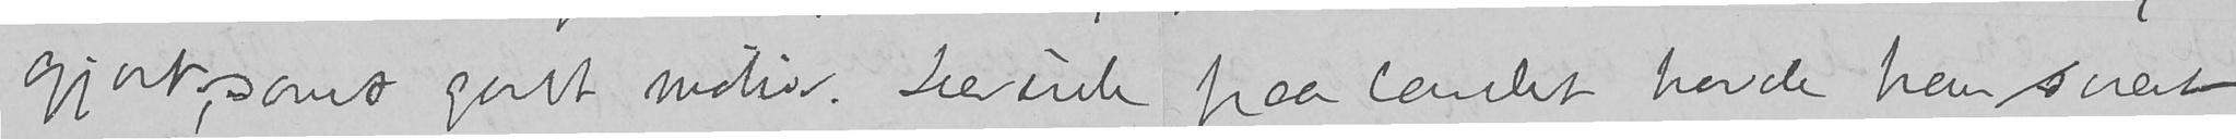gjort, samt godt motiv. Derude paa landet havde han svært
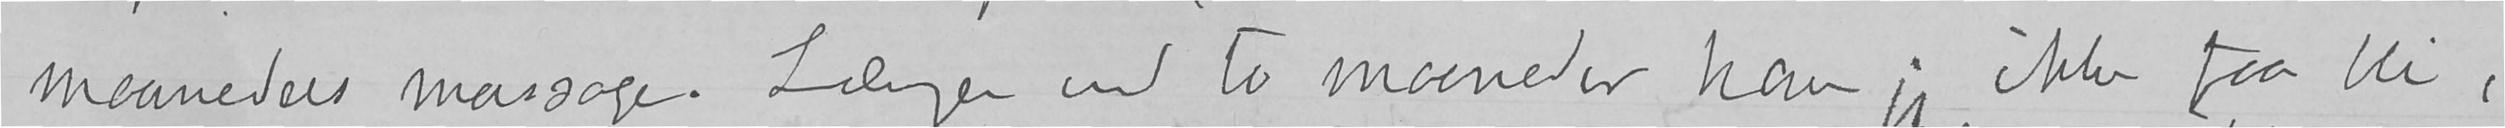maaneders massage. Længer end to maaneder kan jeg ikke faa bli,
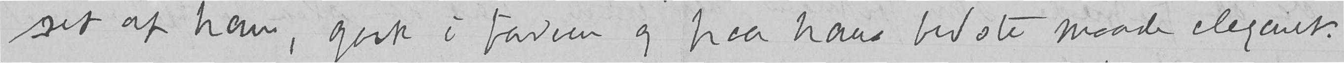set af ham, godt i farven og paa hans bedste maade elegant.
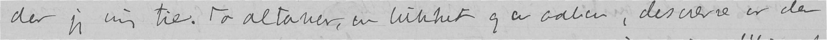der jeg mig til. To altaner, en lukket og en aaben, desværre er der
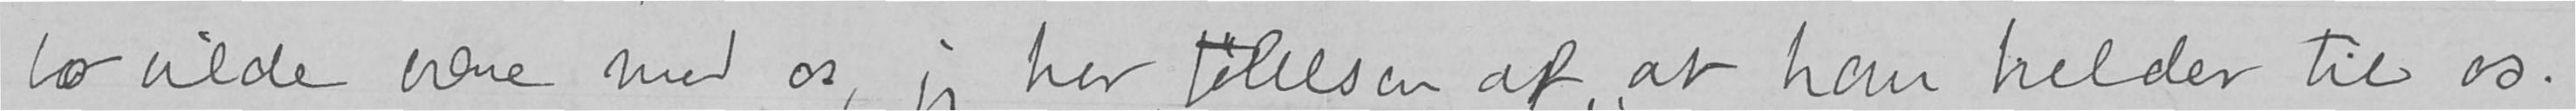bo vilde være med os, jeg har følelsen af, at han helder til os.
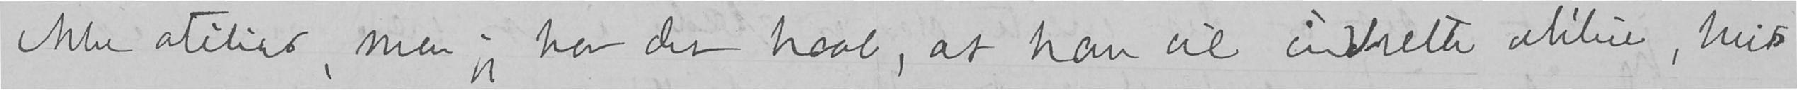ikke atélier, men jeg har det haab, at han vil indrette atélier, hvis
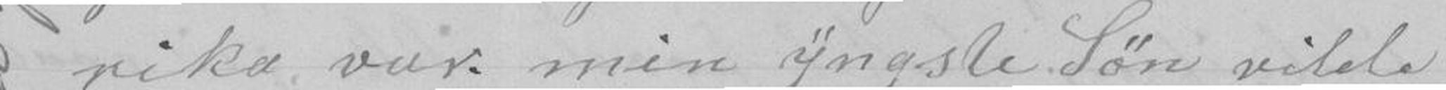rika var min yngste Søn vilde reise
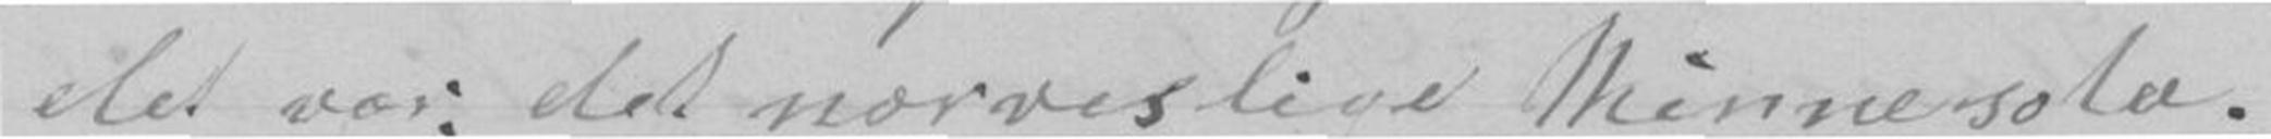det var det norveslige Minnesota.
